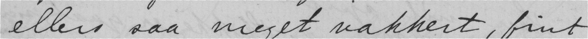ellers saa meget vakkert, fint
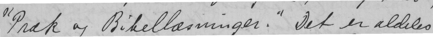"Præk og Bibellæsninger". Det er aldeles
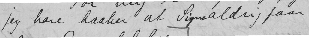jeg bare haaber at Signe aldrig faar
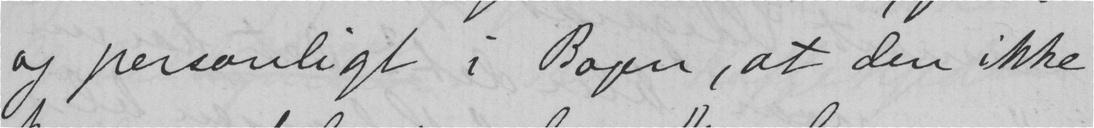og personligt i Bogen, at den ikke
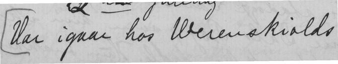Var i gaar hos Werenskiolds
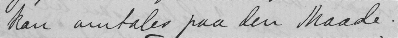kan omtales paa den Maade.
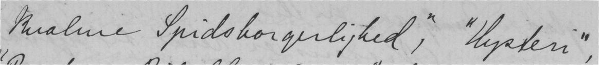kvalme Spidsborgerlighed" "Hysteri",
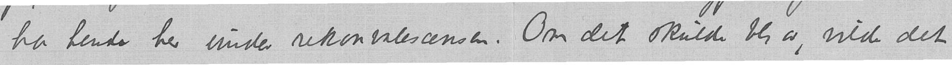ha hende her under rekonvalescensen. Om det skulde bli av, vilde det
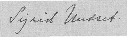Sigrid Undset.
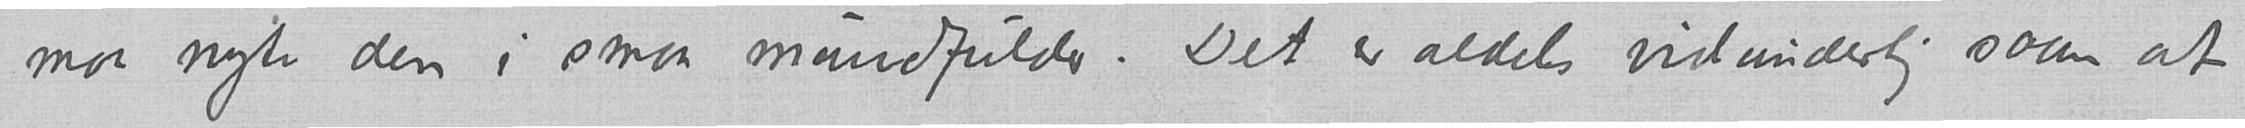maa nyte den i smaa mundfulder. Det er aldeles vidunderlig saan at
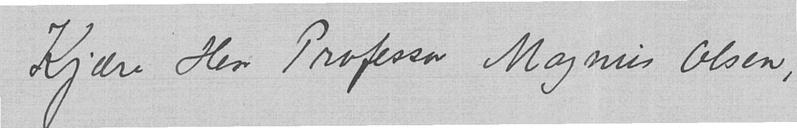Kjære Herr Professor Magnus Olsen,
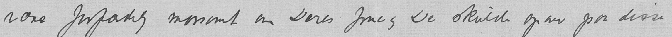være forfærdelig morsomt om Deres frue og De skulde opover paa disse
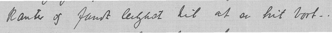kanter og fandt leilighet til at se hit bort –.
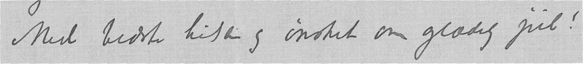Med bedste hilsen og ønsket om glædig jul!
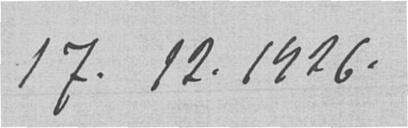17.12.1926.
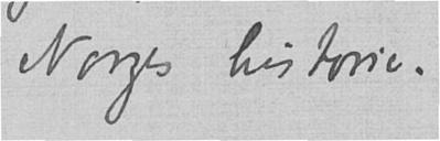Norges historie.
